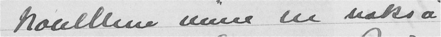Novellene mine en nokså
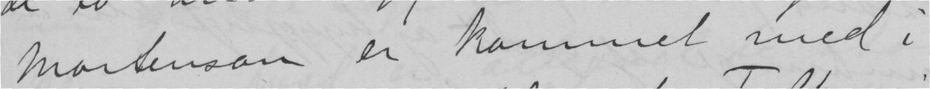Mortenson er kommet med i
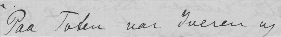Paa Toten var Iveren og
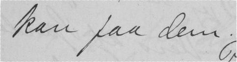kan faa dem.
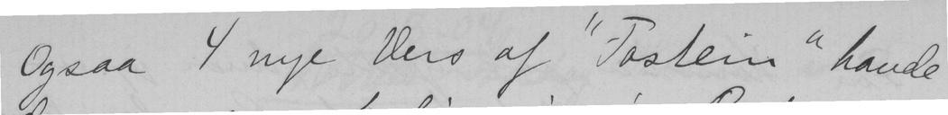Ogsaa 4 nye Vers af "Tostein" havde
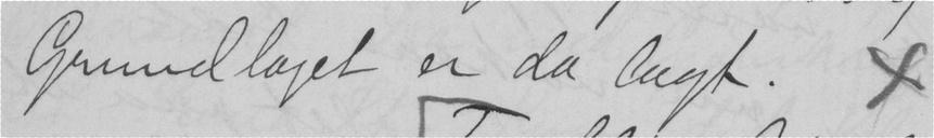Grundlaget er da lagt. x
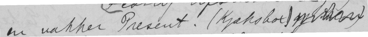en vakker Present. (Kjæksbot)
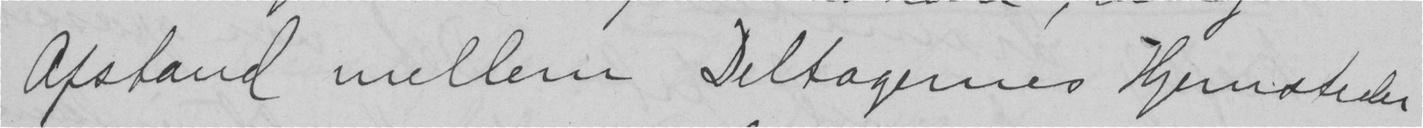Afstand mellem Deltagernes Hjemsteder
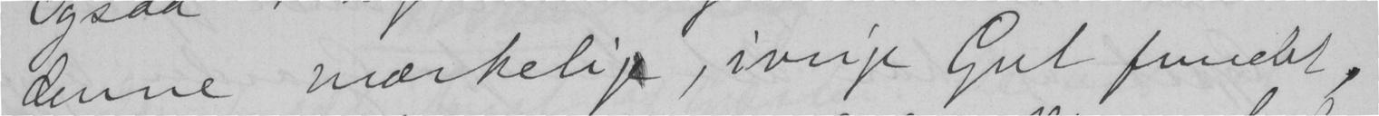denne mærkelig, ivrige Gut fundet,
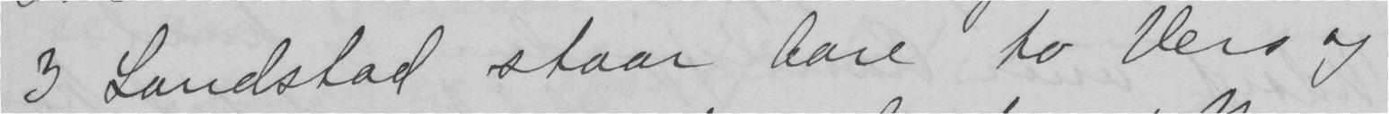I Landstad staar bare to Vers og
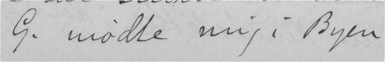G. mødte mig i Byen
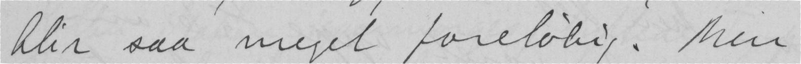blir saa meget foreløbig. Men
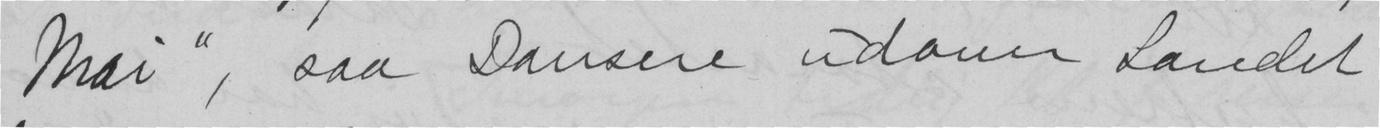Mai", saa Dansere udover Landet
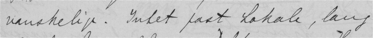vanskelige. Intet fast Lokale, lang
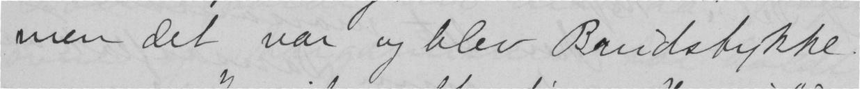men det var og blev Brudstykke.
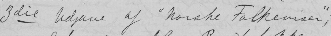3die Udgave af "norske Folkeviser";
